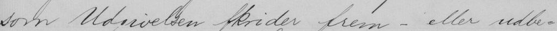som Udgivelsen skrider frem - eller udbe-
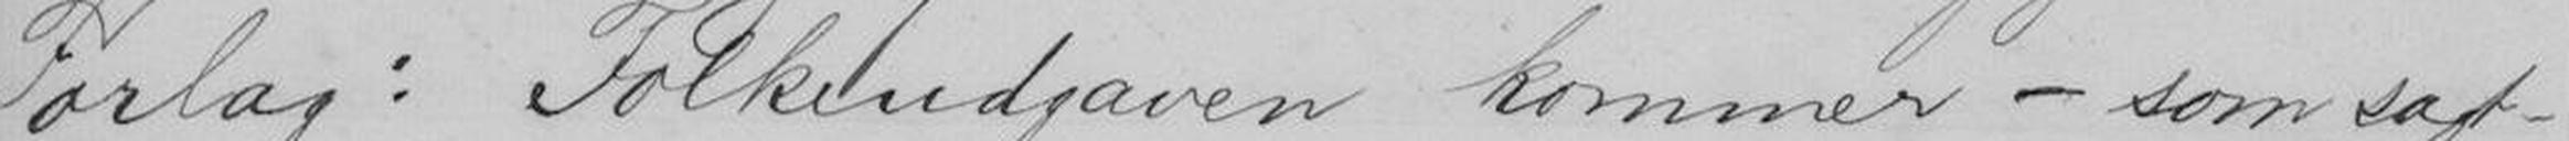Forlag: Folkeudgaven kommer - som sagt -
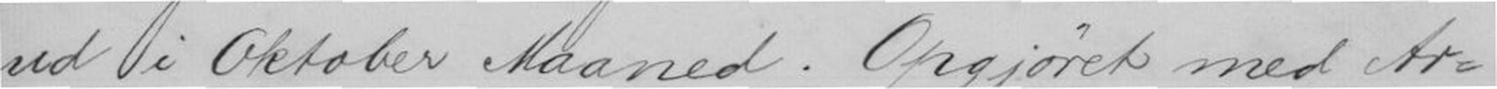ud i Oktober Maaned. Opgjøret med Ar-
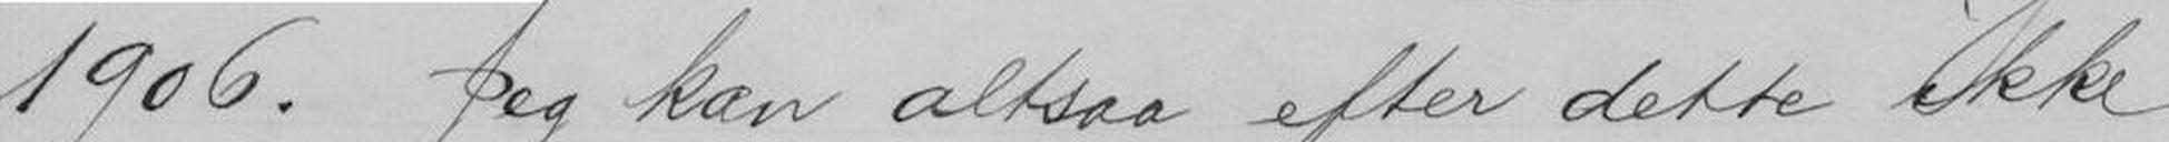1906. Jeg kan altsaa efter dette ikke
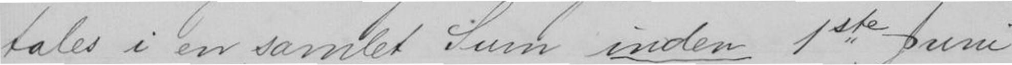tales i en samlet Sum inden 1ste Juni
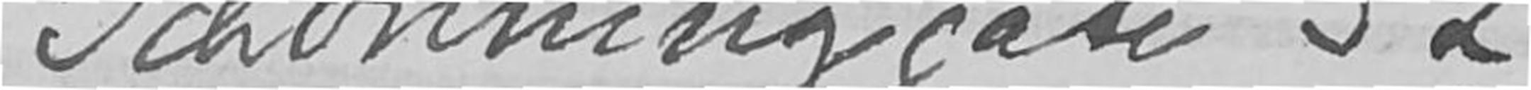Schonningsgate 32II
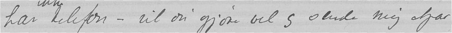har ikke telefon – vil du gjøre vel og sende mig etpar
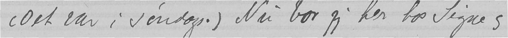(Det var i søndags.) Nu bor jeg her hos Signe og
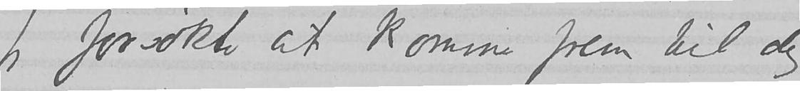Jeg forsøkte at komme frem til dig
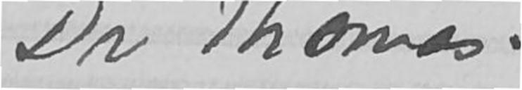Dr. Thomas
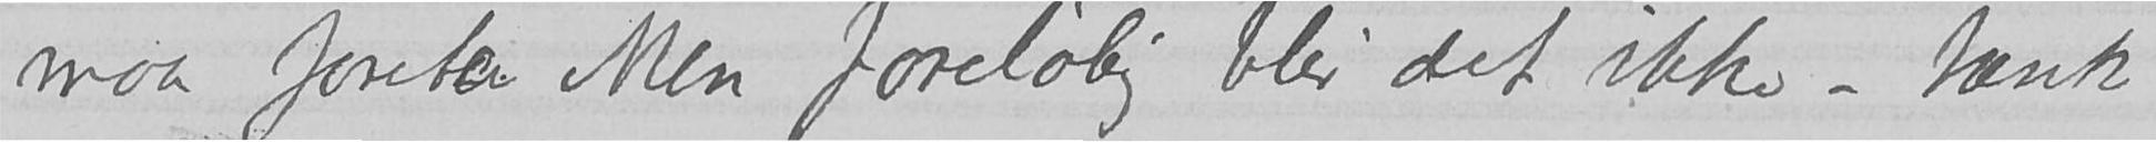maa foretas. Men foreløpig blir det ikke –tænk
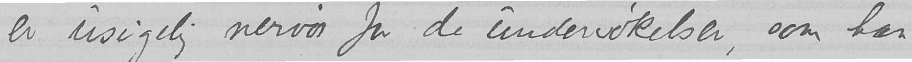er usigelig nervøs for de undersøkelser, som her
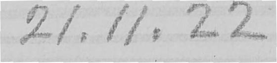21.11.22
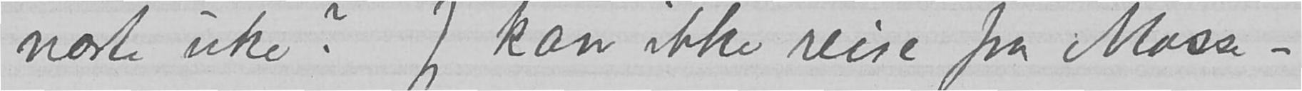næste uke? Jeg kan ikke reise fra Mosse –
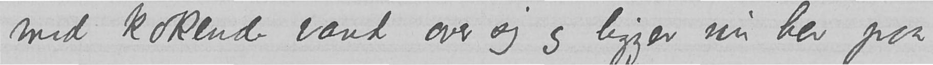med kokende vand over sig og ligger nu her paa
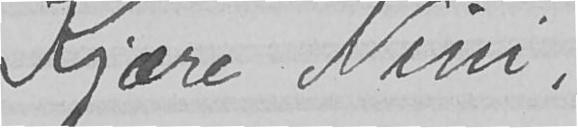Kjære Nini
